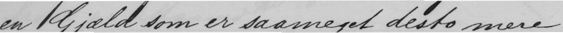en Gjæld som er saameget desto mere
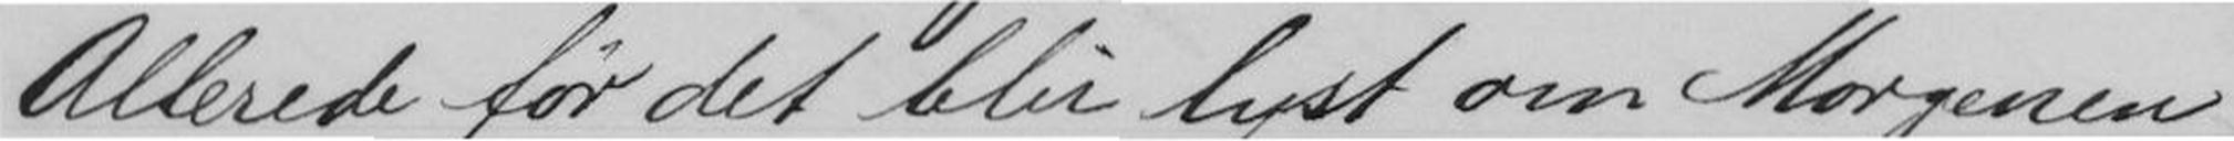Allerede før det blir lyst om Morgenen
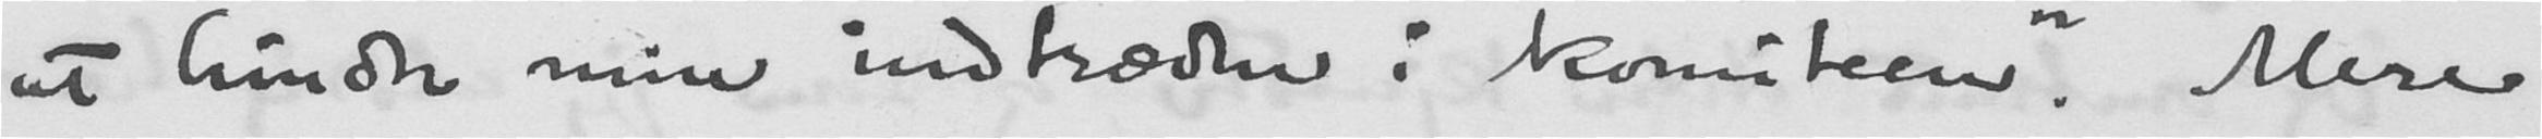at hindre min indtræden i komiteen". Mere
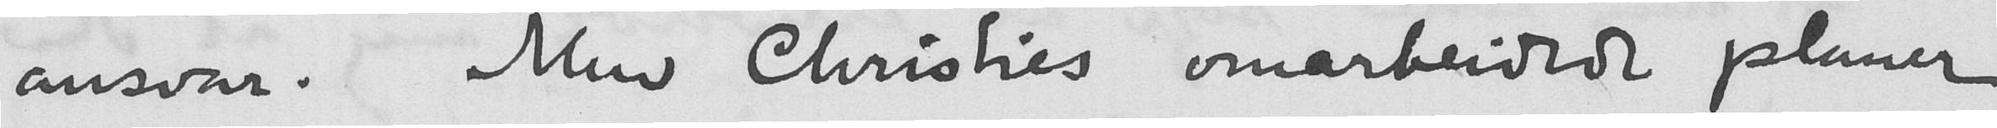ansvar. Men Christies omarbeidede planer
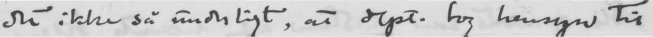det ikke så underligt, at dept. tog hensyn til
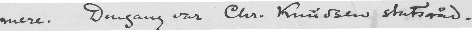mere. Dengang var Chr. Knudsen statsråd.
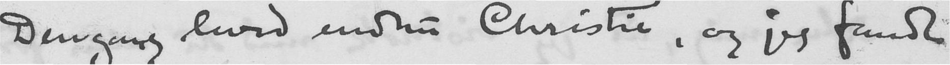Dengang leved endnu Christie, og jeg fandt
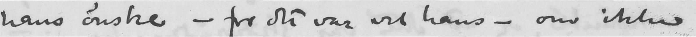hans ønske - for det var vel hans - om ikke
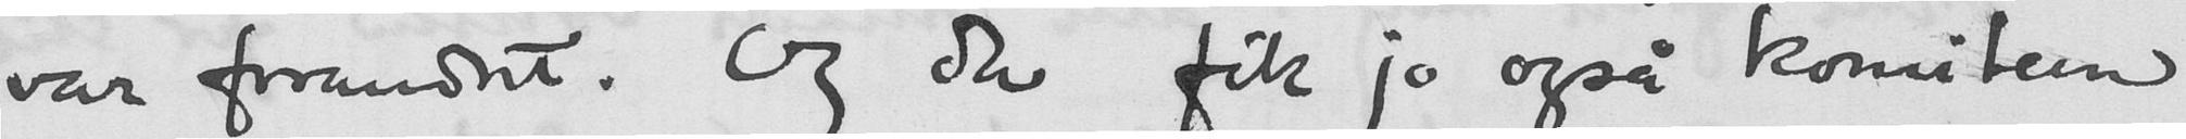var forandret. Og da fik jo også komiteen
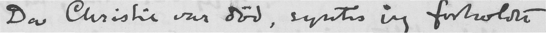Da Christie var død, syntes jeg forholdet
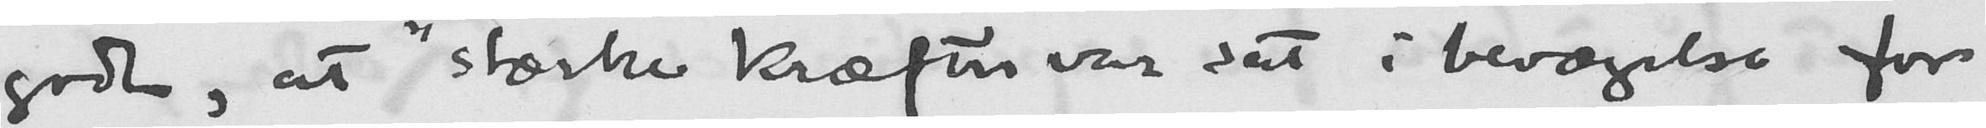godt, at "stærke kræfter vat sat i bevægelse for
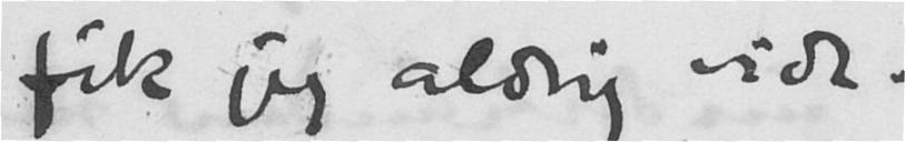fik jeg aldrig vide.
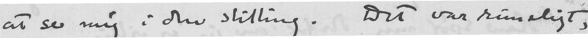at se mig i den stilling. Det var rimeligt,
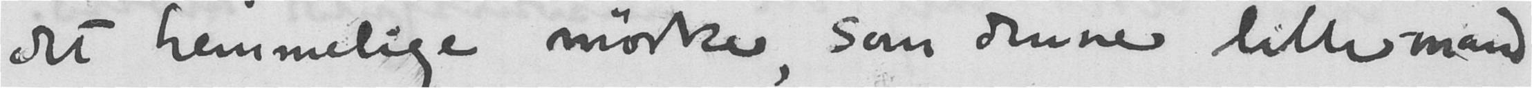det hemmelige mørke, som denne lille mand
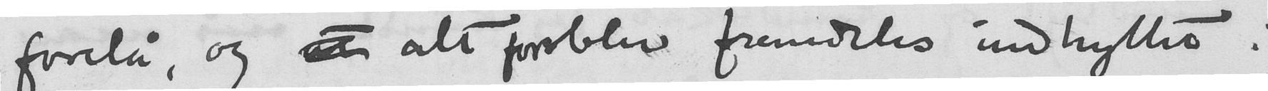forelå, og at alt forble framdeles indhyllet i
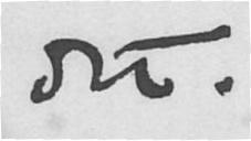det.
